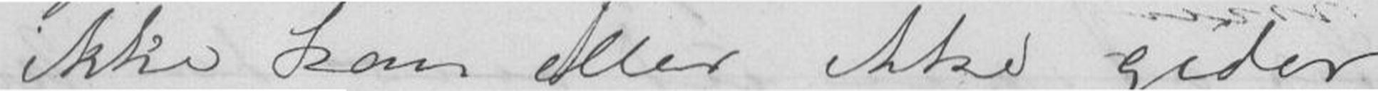ikke kan eller ikke gider
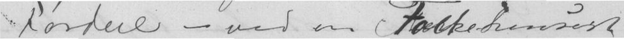Fordell - ved en Folkekonsert
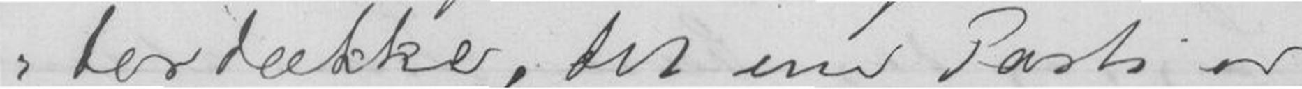der dække, det ene Parti er
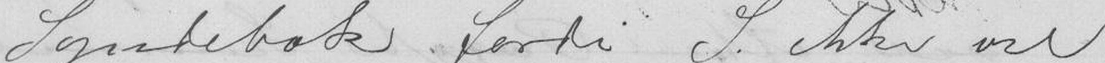Syndebok fordi S. ikke vil
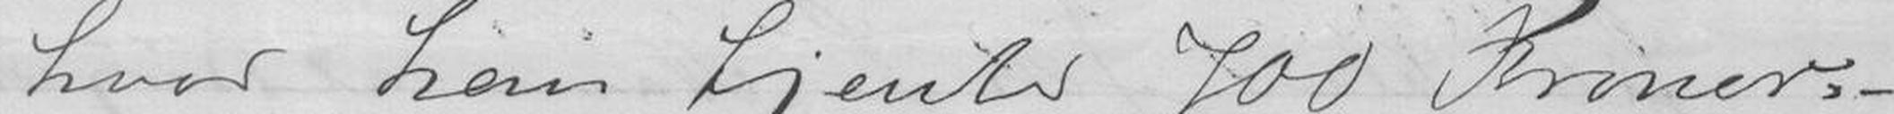hvor han tjente 700 Kroner. -
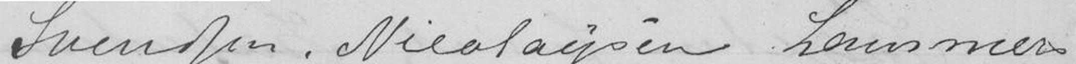Svendsen, Nicolaysén Lammers
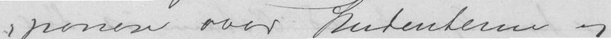ponere over Studenterne og
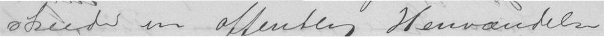skeede en offentlig Henvændelse
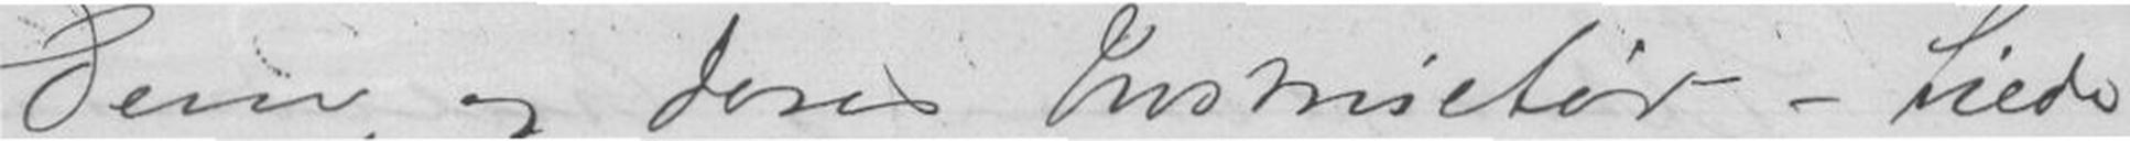Dem og Deres Indtructør - tiede
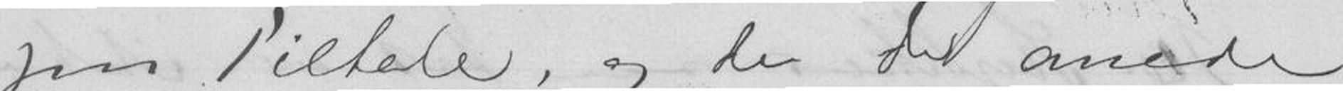paa Tiltale, og da de anede
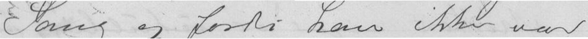Sang og fordi han ikke var
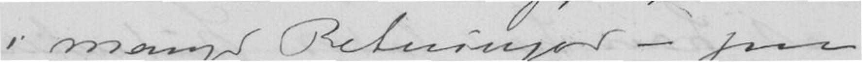i mange Retninger - paa
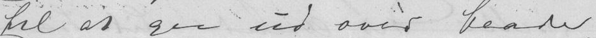til at gaa ud over baade
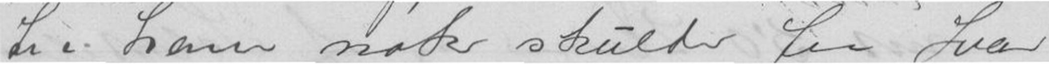til ham nok skulde faa Svar
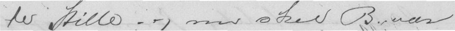de Stille og nu skal B. være
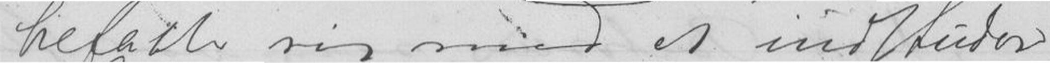befatte sig med at indstudere
